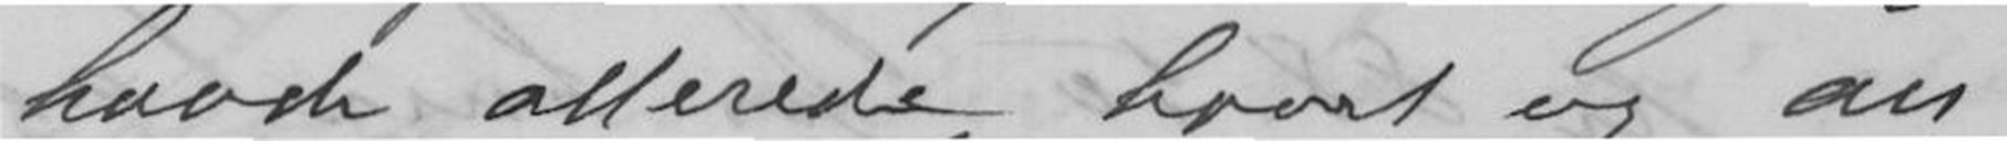havde allerede hævet og an-
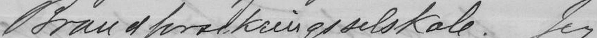Brandforsikringselskab. Jeg
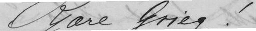Kjære Grieg!
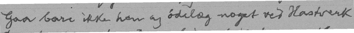Gaa bare ikke hen og ødelæg noget ved Hastverk
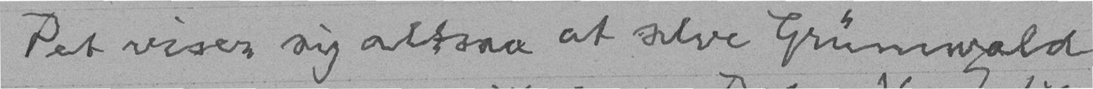Det viser sig altsaa at selve Grünewald
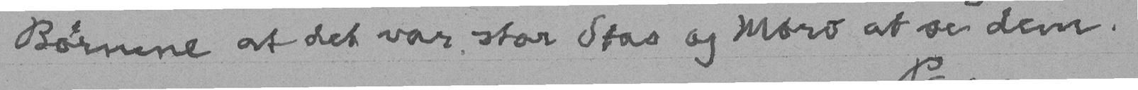Børnene at det var stor Stas og Moro at se dem.
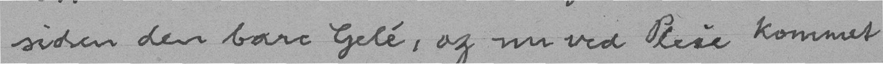siden den bare Gelé, og nu ved Pleie kommet
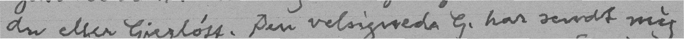du eller Gierløff. Den velsignede G. har sendt mig
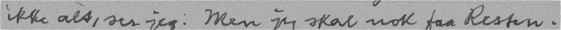ikke alt, ser jeg. Men jeg skal nok faa Resten.
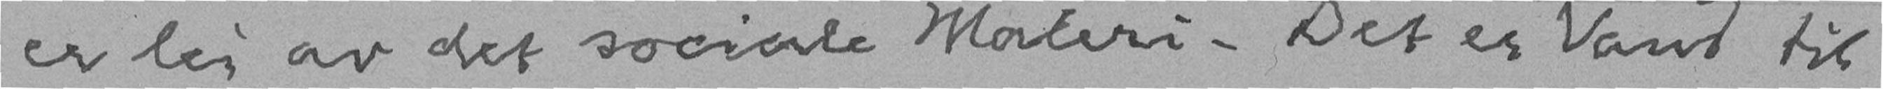er lei av det sociale Maleri. Det er Vand til
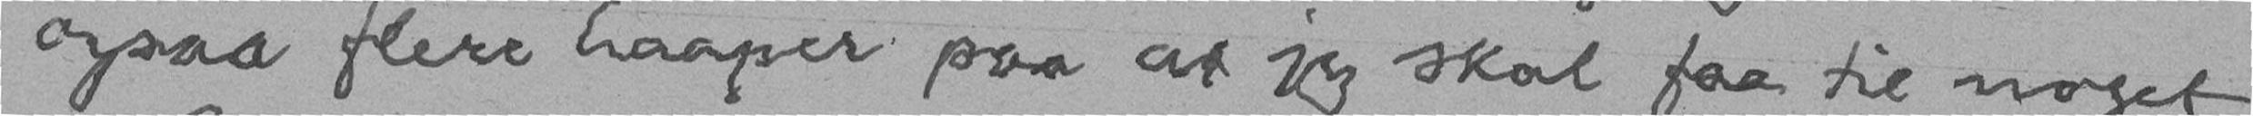ogsaa flere haaper paa at jeg skal faa til noget
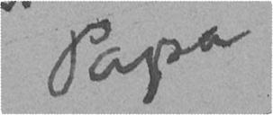Papa
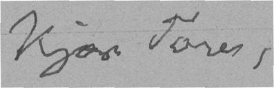Kjære Tore,
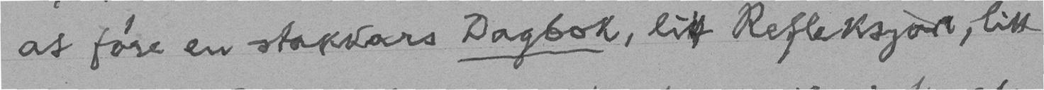at føre en stakkars Dagbok, litt Refleksjon, litt
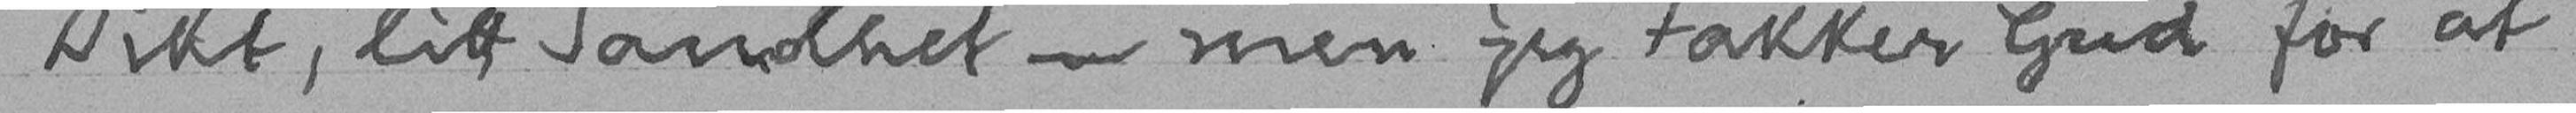Dikt, litt Sandhet - men jeg takker Gud for at
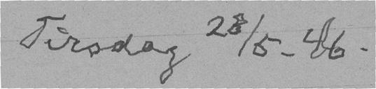Tirsdag 28/5. 46.
